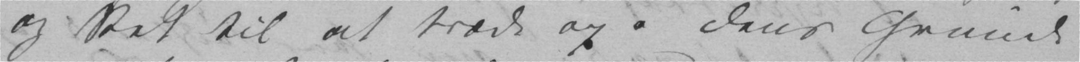og Ret til at træde op. Dens Grunde
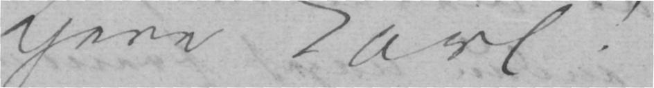Kjere Carl!
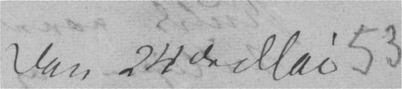Den 24de Mai
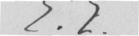C.C.
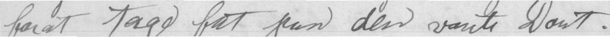forat tage fat paa den vante Dont.
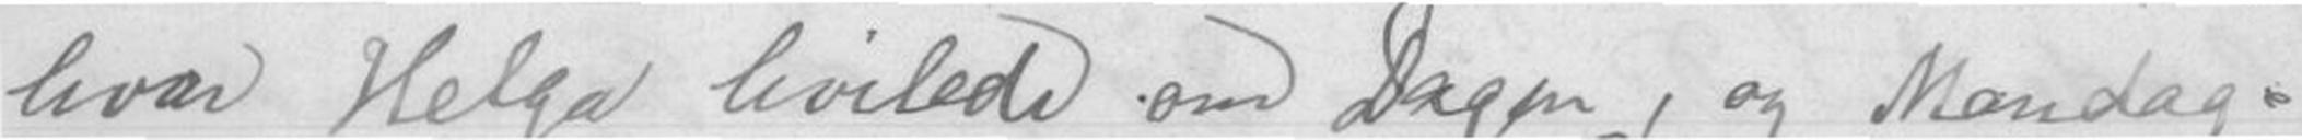hvor Helga hvilede om Dagen, og Mandag-
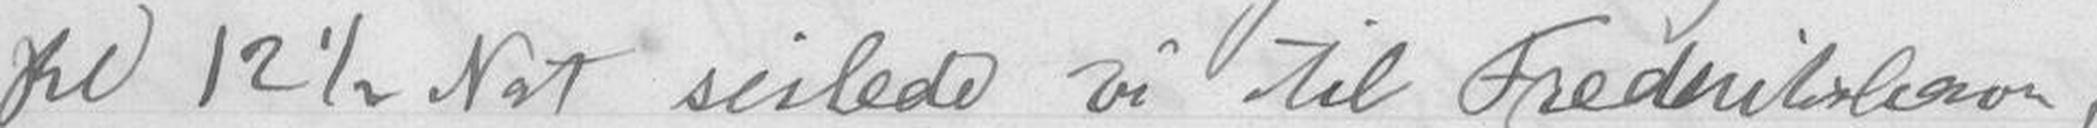Kl 12 1/2 Nat seilede vi til Fredrikshavn,
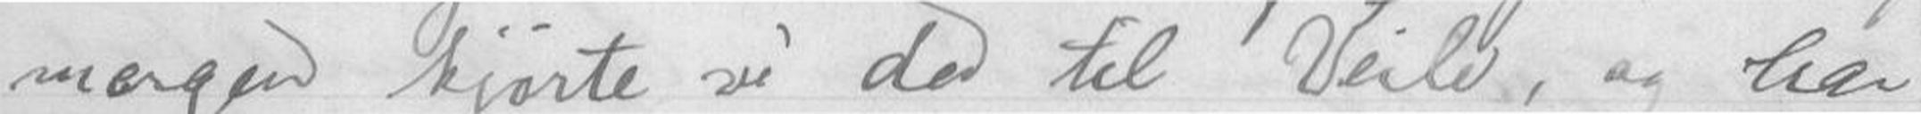morgen kjørte vi da til Veile, vi har
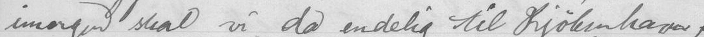imorgen skal vi da endelig til Kjøbenhavn,
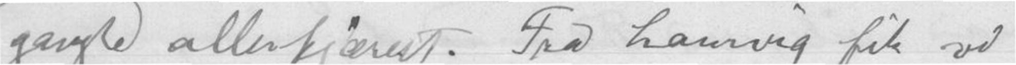ganske allerkjærest. Fra Laurvig fik vi
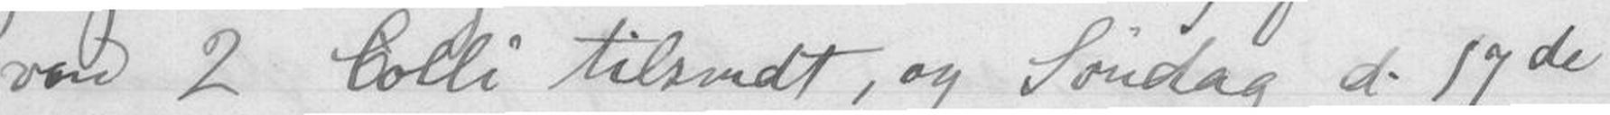vore 2 Colli tilsendt, og Søndag d. 17de
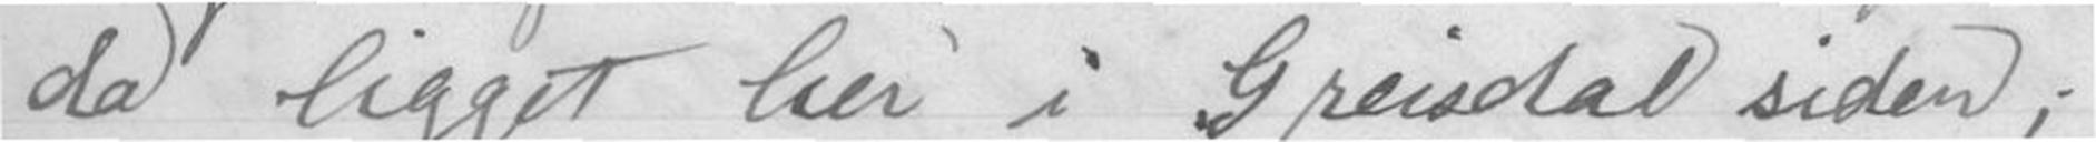da ligget her i Greisdal siden;
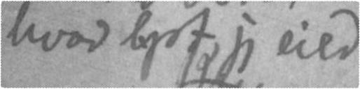hvad lyst jeg eied
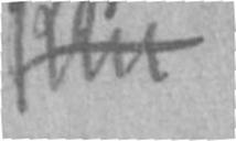Hlu
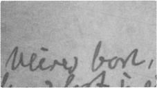veired bort,
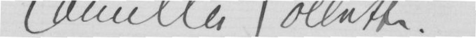Camilla Collett.
